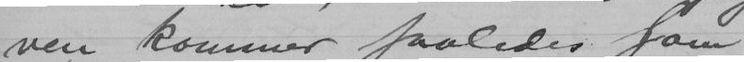ven kommer saaledes som
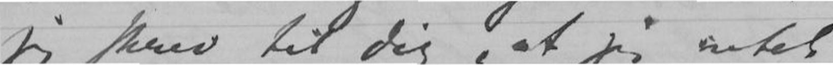jeg skrev til dig, at jeg intet
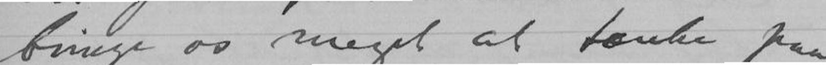bringe os meget at tænke paa
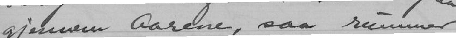gjennem Aarene, saa rummer
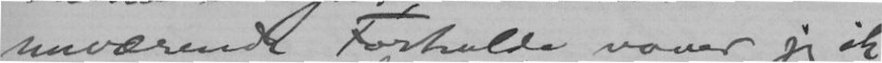nuværende Forholde vover jeg ik-
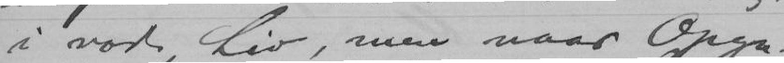i vort Liv, men naar Opga-
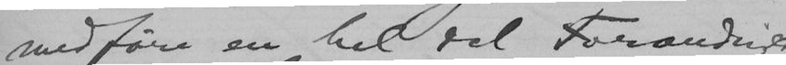medføre en hel del Forandringer
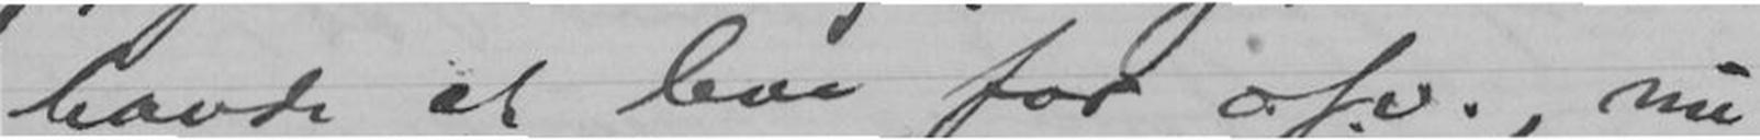havde at leve for osv., nu
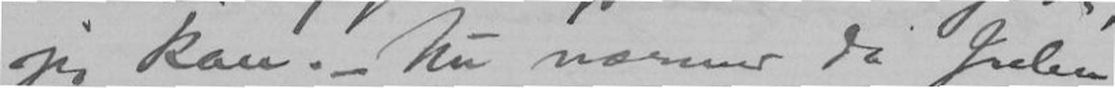jeg kan. - Nu nærmer da Julen
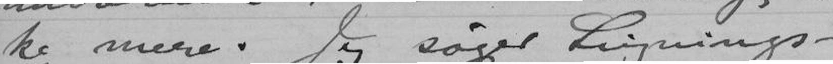ke mere. Jeg søger Lignings-
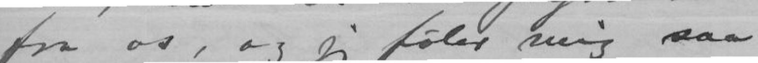fra os, og jeg føler mig saa
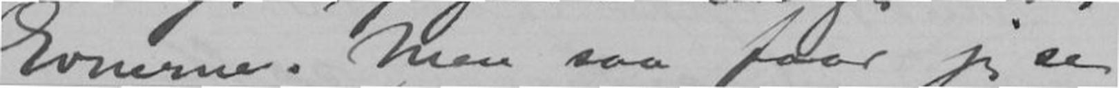Evnerne. Man saa faar jeg en
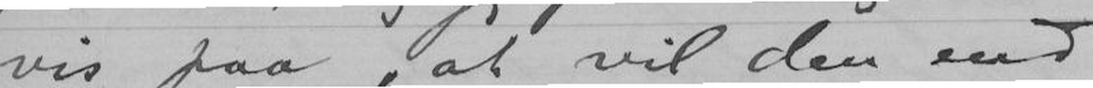vis paa, at vil den end
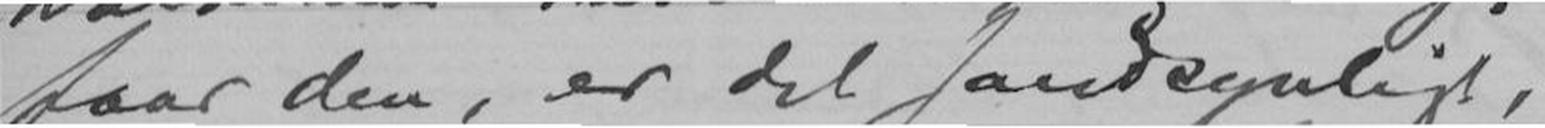faar den, er det sandsynligt,
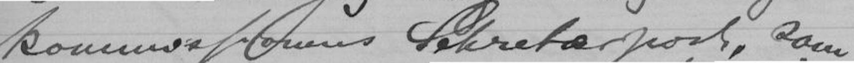kommissonens Sekretærpost, som
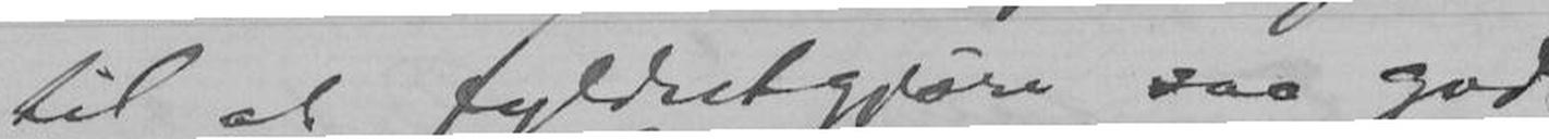til at fyldestgjøre saa godt,
